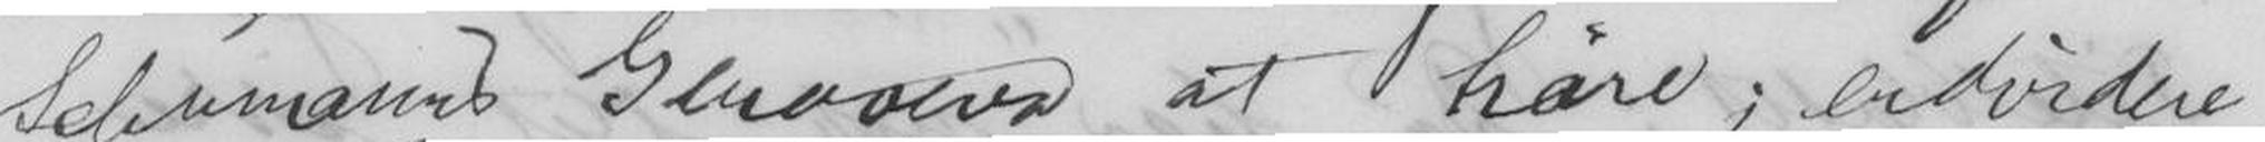Schumann Genoveva at høre; endvidere
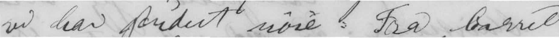vi har studert nøie. Fra Cassel
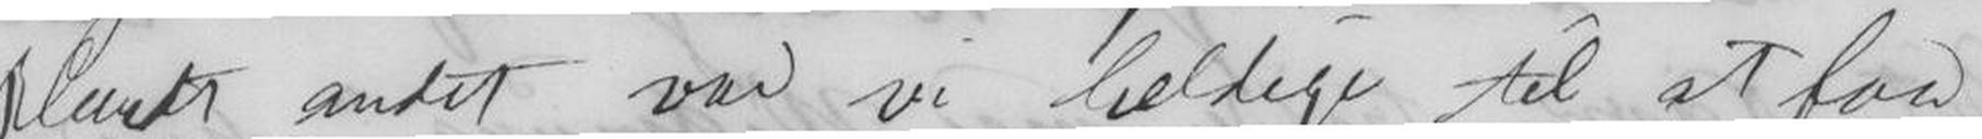Blandt andet var vi heldige til at faa
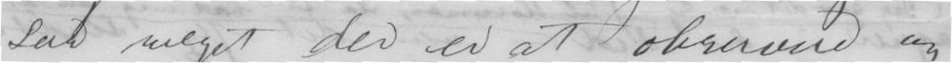saa meget der er at observere og
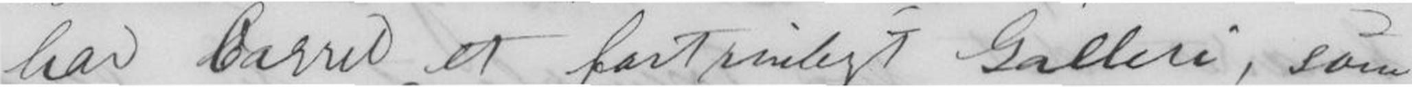har Cassel et fortrinligt Galleri, som
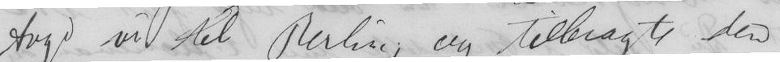tog vi til Berlin; og tilbragte den
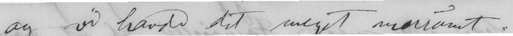og vi havde det meget morsomt.
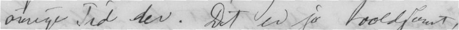øvrige Tid der. Det er jo voldsomt,
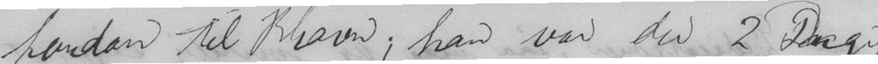London til Khavn; han var der 2 Dage,
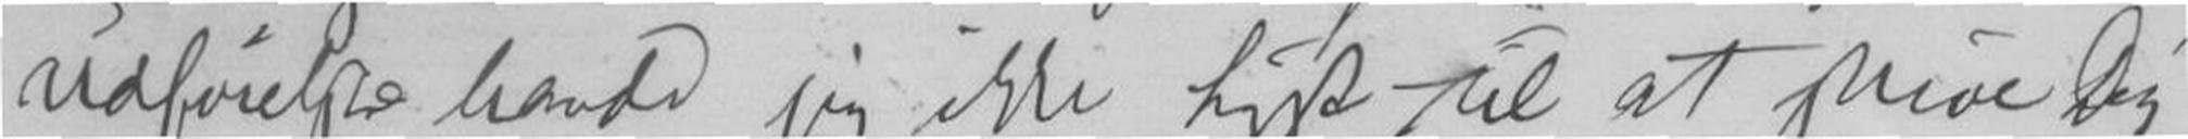Udførelse havde jeg ikke Lyst til at skrive Dig
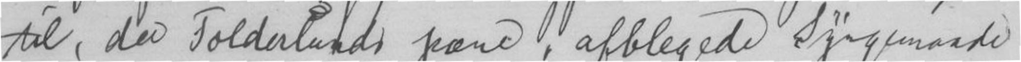til, da Foldelunds pæne, afblegede Syngemaade
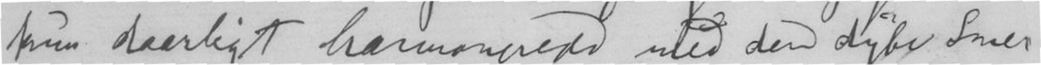kun daarligt harmonerende med den dybe Smer-
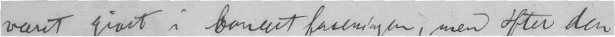været givet i Concertforeningen, men efter den
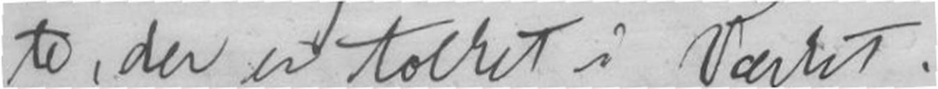te, der er tolket i Værket.
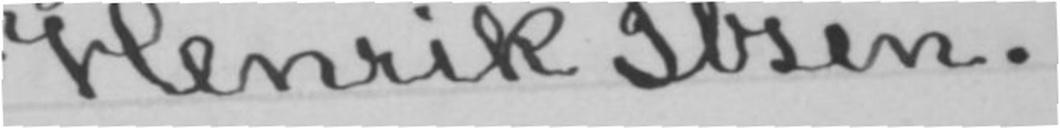Henrik Ibsen.
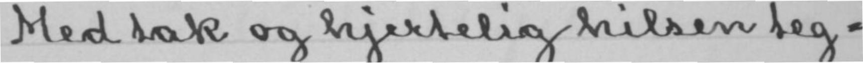Med tak og hjertelig hilsen teg-
